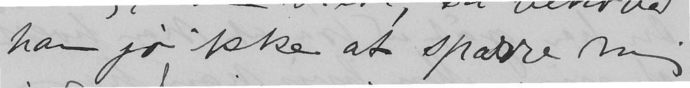han jo ikke at spærre meg
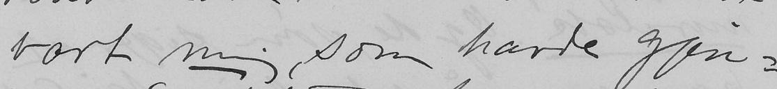vært mig, som havde gjen-
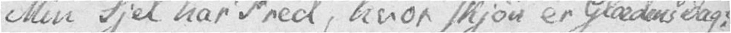Min Sjel har Fred, hvor skjøn er Glædens Dag:
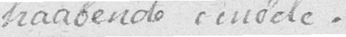haabende imøde.
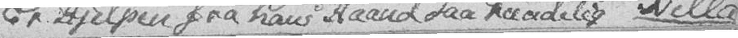Er Hjelpen fra hans Haand saa raadelig Nella
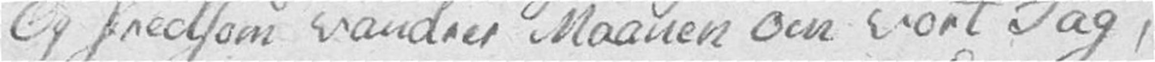Og fredsom vandrer Maanen om vort Tag,
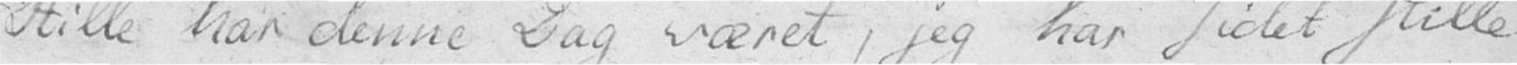Stille har denne Dag været, jeg har sidet stille
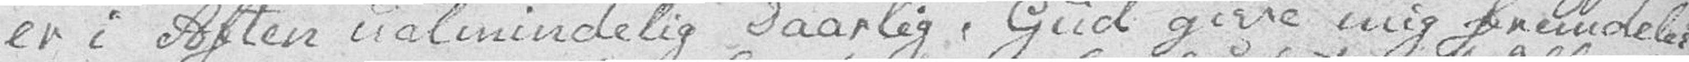er i Aften ualmindelig Daarlig. Gud give mig fremdeles
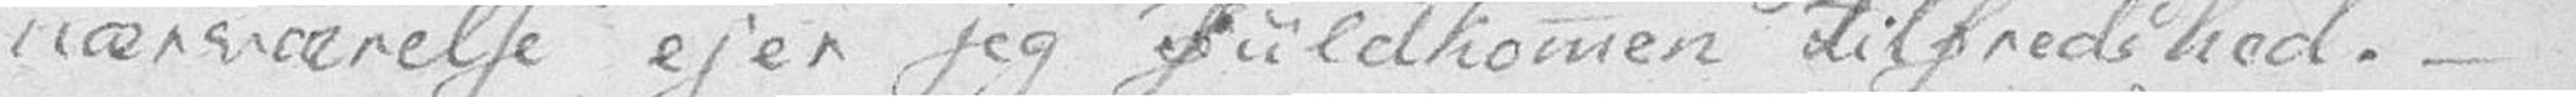nærværelse ejer jeg Fuldkommen Tilfredshed. –
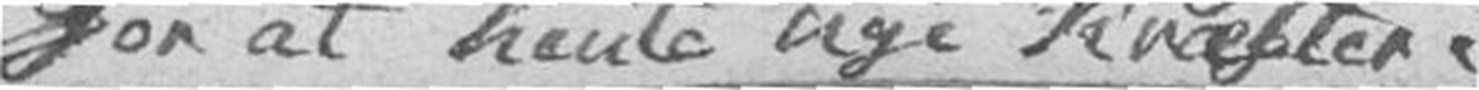for at hente nye Kræfter.
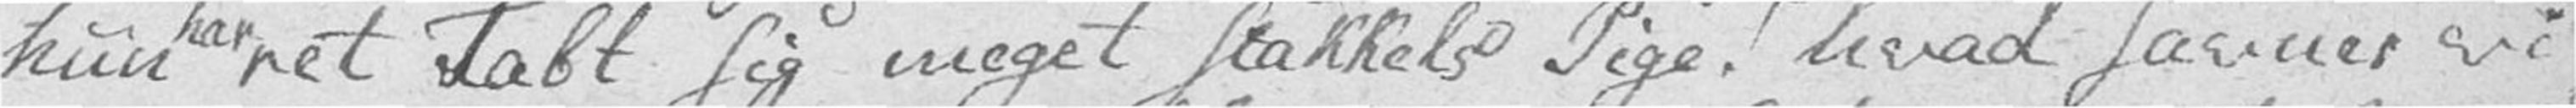hun har ret Tabt sig meget stakkels Pige! hvad savner vi
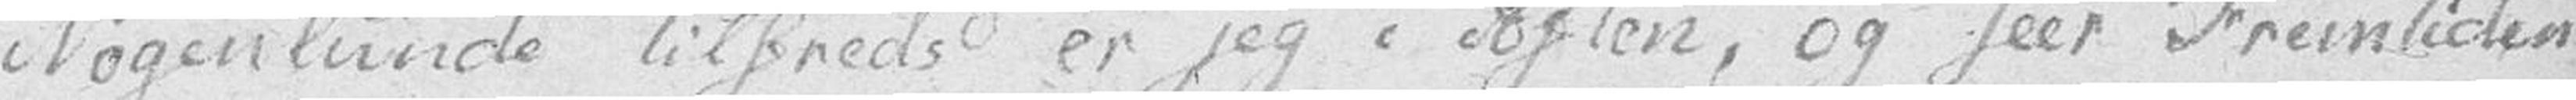Nogenlunde tilfreds er jeg i Aften, og seer Fremtiden
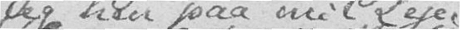jeg hen paa mit Leje
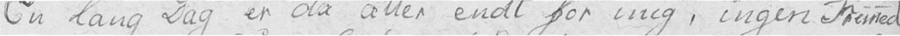En lang Dag er da atter endt for mig, ingen Fremmed
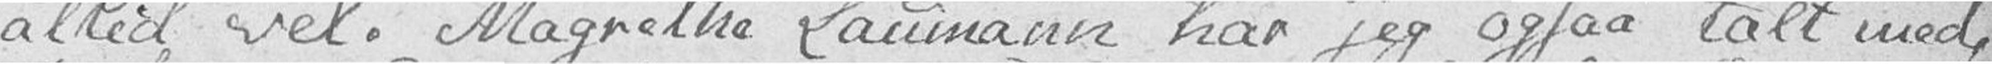altid vel. Margrethe Laumann har jeg ogsaa talt med,
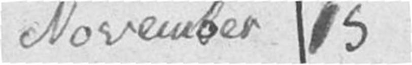November 15
